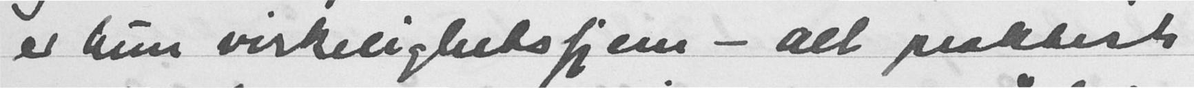er hun virkelighetsfjern - alt praktisk
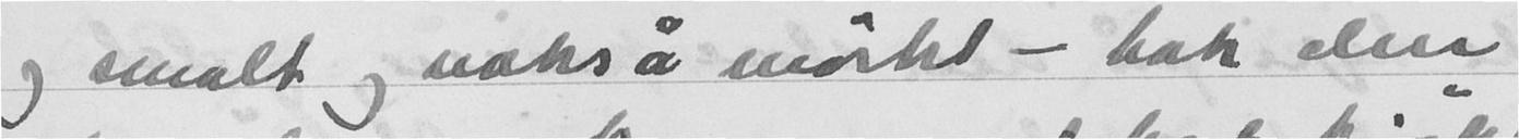og smalt og nokså mørkt - bak den
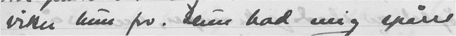viker hun for. Hun bad mig spørre
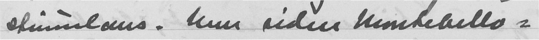stimulans. Men siden Montebello-
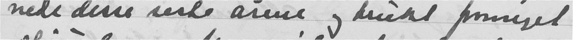nede disse siste årene og brukt formeget
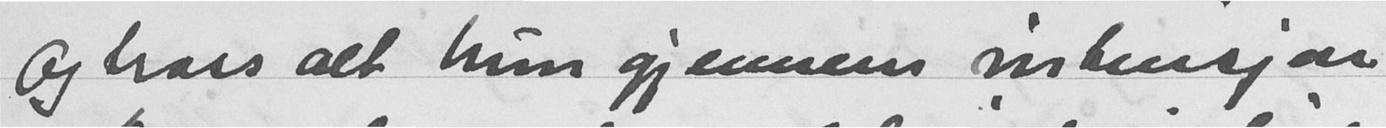Og tross alt hun gjennom intensjon
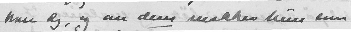hver dag, og om dem snakker hun som
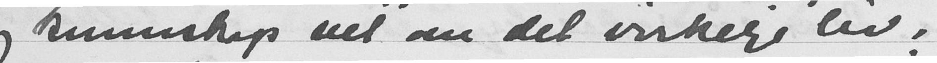og kunnskap vet om det virkelige liv,
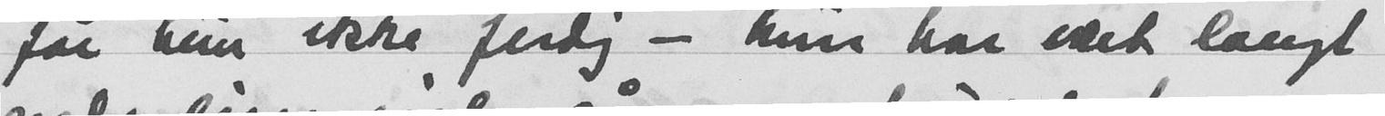får hun ikke ferdig - hun har vært langt
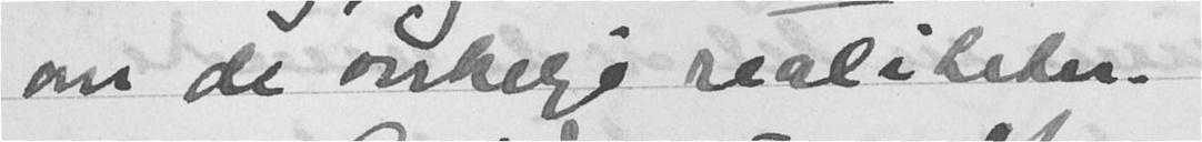om de virkelige realiteter.
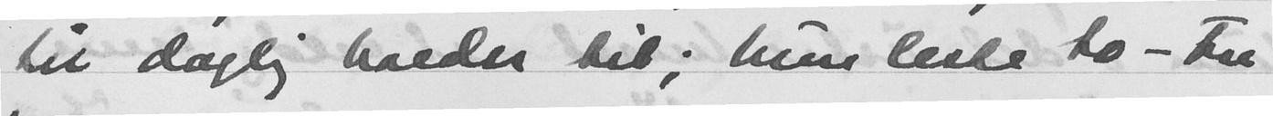til daglig holder til; hun leste to-tre
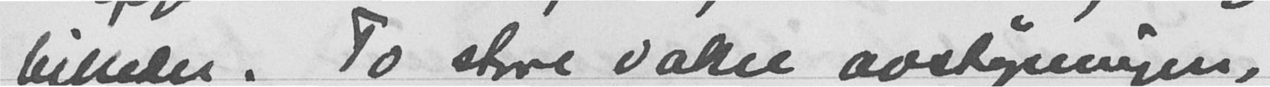billeder. To store vakre avstøpninger,
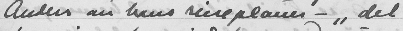Anders om hans reiseplaner - "det
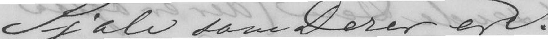Sjæle som Derer er.
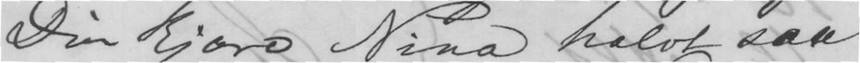Din kjære Nina halvt saa
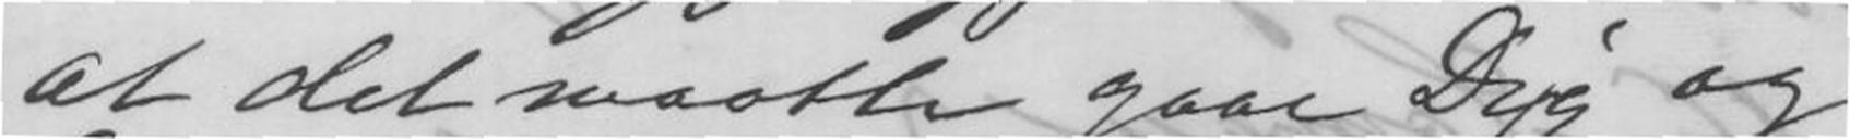at det maatte gaae Dig og
